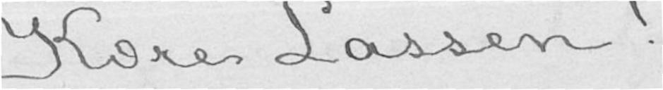Kære Lassen!
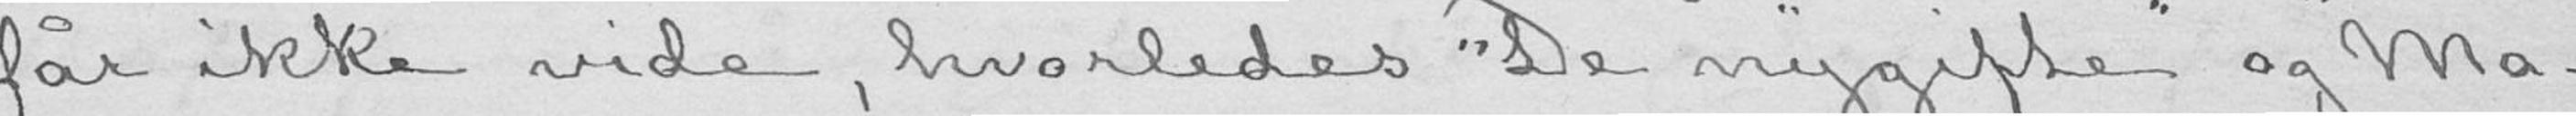får ikke vide, hvorledes «De nygifte» og «Ma-
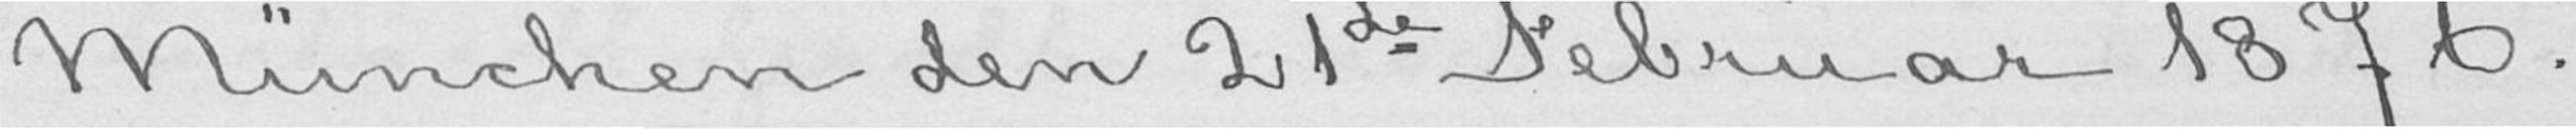München den 21de Februar 1876.
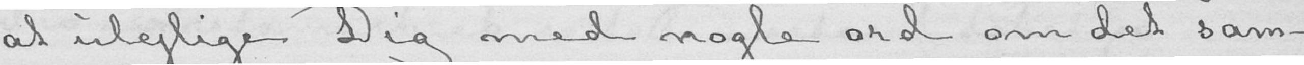at ulejlige Dig med nogle ord om det sam-
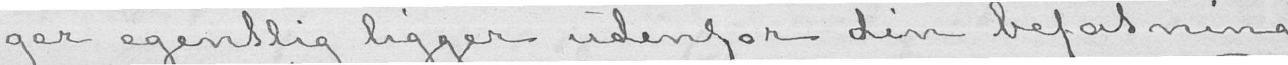ger egentlig ligger udenfor din befatning
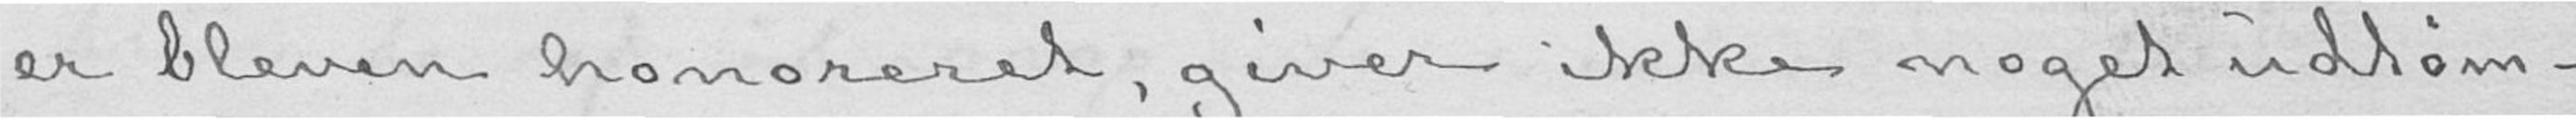er bleven honoreret, giver ikke noget udtøm-
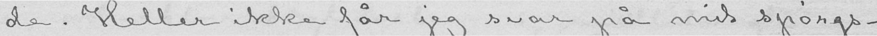de. Heller ikke får jeg svar på mit spørgs-
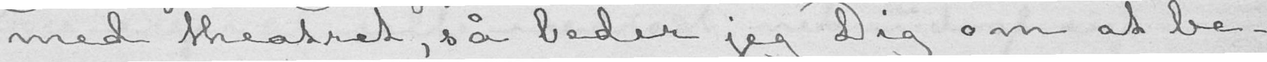med theatret, så beder jeg Dig om at be-
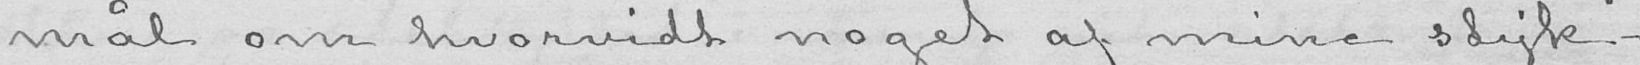mål om hvorvidt noget af mine styk-
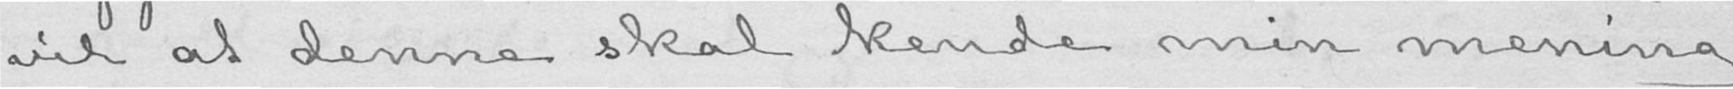vil at denne skal kende min mening
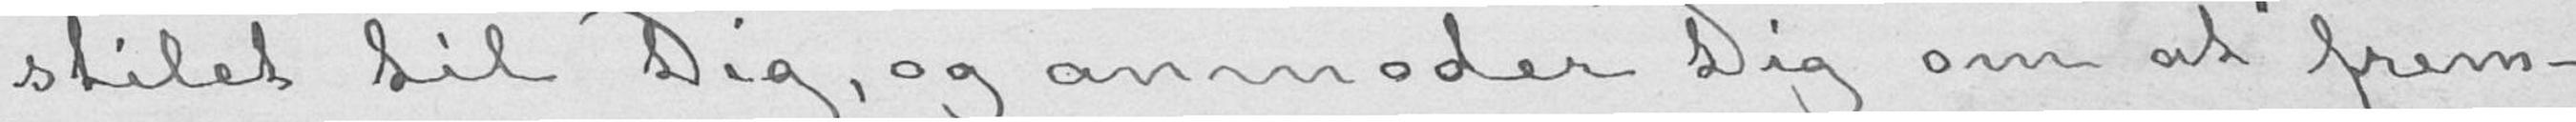stilet til Dig, og anmoder Dig om at frem-
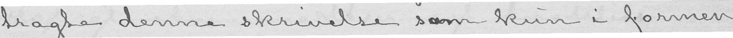tragte denne skrivelse som kun i formen
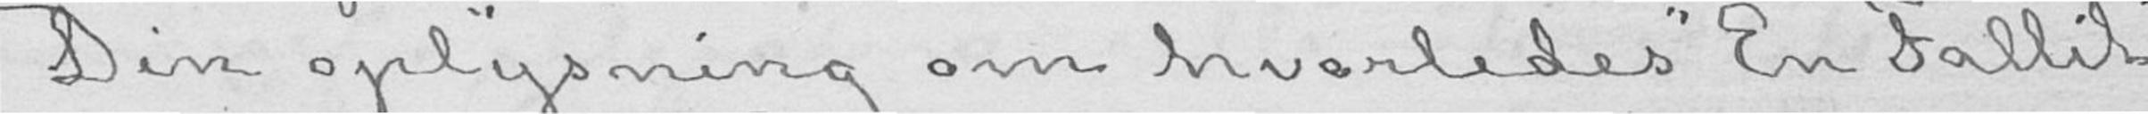Din oplysning om hvorledes «En Fallit»
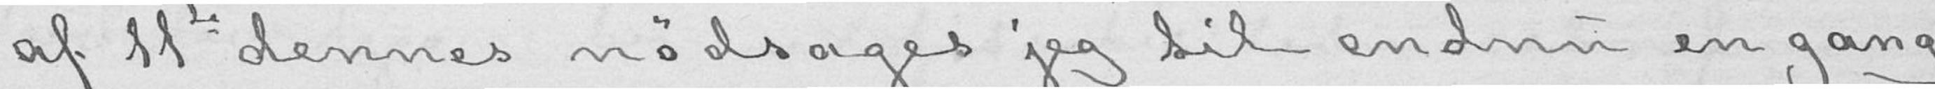af 11te dennes nødsages jeg til endnu en gang
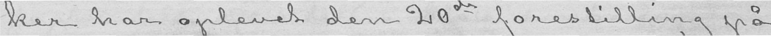ker har oplevet den 20de forestilling på
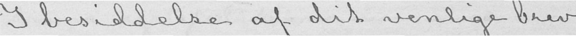I besiddelse af dit venlige brev
